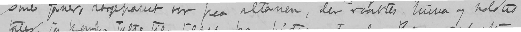sine faner, Kongeparret var paa altanen, der raabtes hurra og holdtes
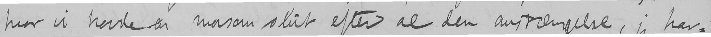hvor vi havde en morsom slut efter al den anstrængelse, jeg hav-
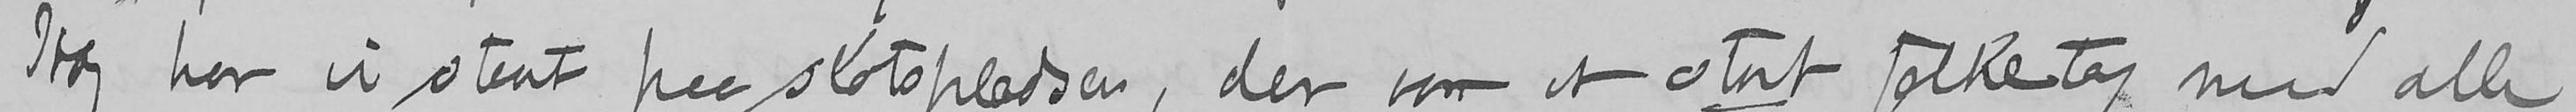Idag har vi staat paa slotspladsen, der var et stort folketog med alle
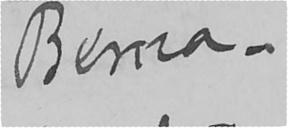Berna-
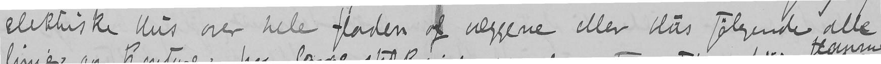elektrisk blus over hele fladen af væggene eller blus følgende alle
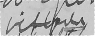viftede
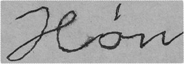Høn
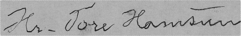Hr. Tore Hamsun
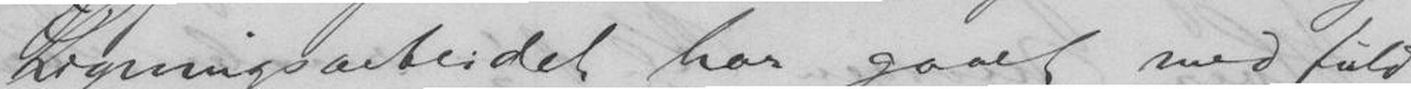Ligningsarbeidet har gaaet med fuld
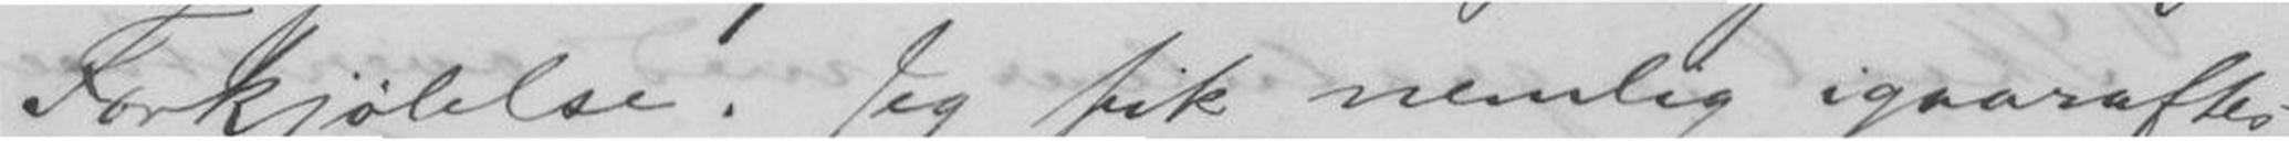Forkjølelse. Jeg fik nemlig igaaraftes
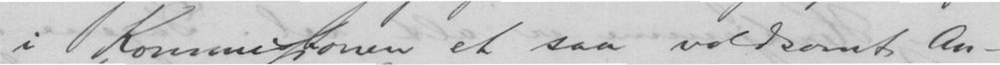i Kommisionen et saa voldsomt An-
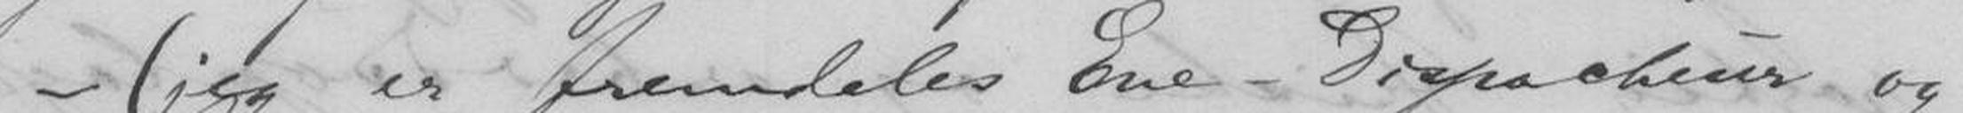- (jeg er fremdeles Ene-Dispacheur og
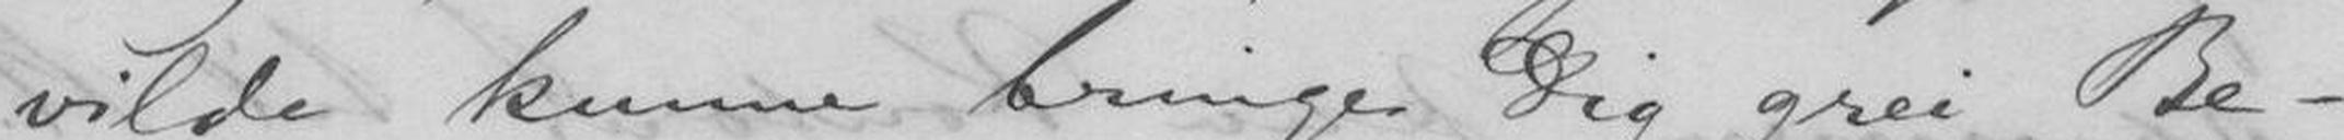vilde kunne bringe Dig grei Be-
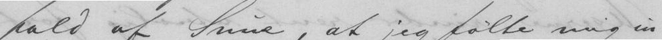fald af Snue, at jeg følte mig in-
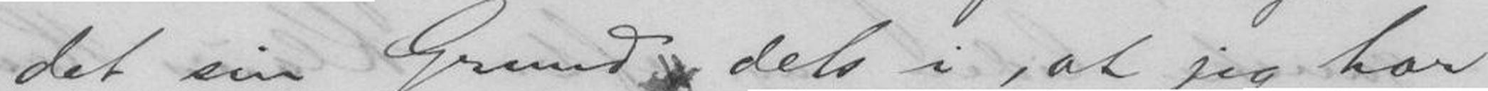det sin Grund , dels i, at jeg har
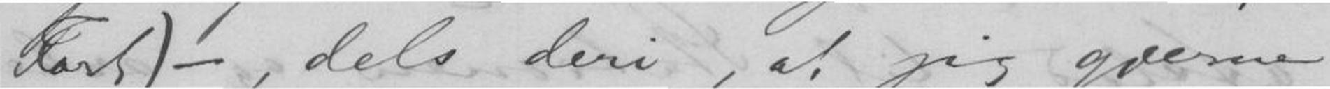Fart) -, dels deri, at jeg gjerne
